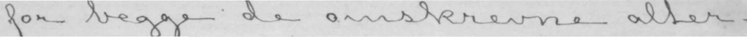for begge de omskrevne alter-
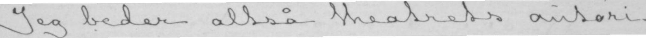Jeg beder altså theatrets autori-
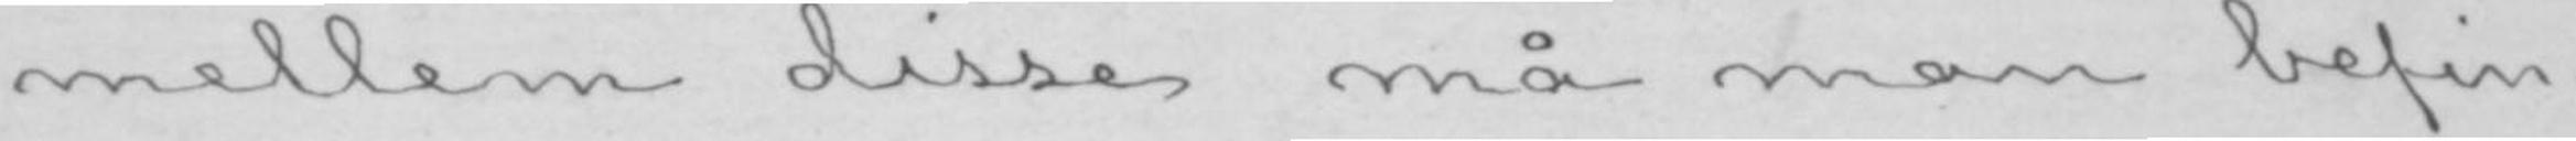mellem disse må man befin-
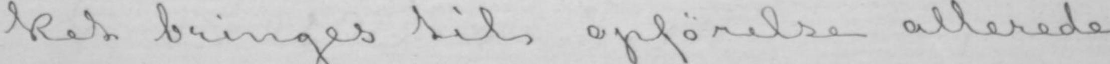ket bringes til opførelse allerede
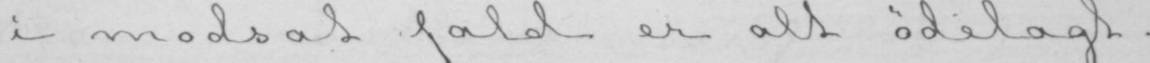i modsat fald er alt ødelagt.
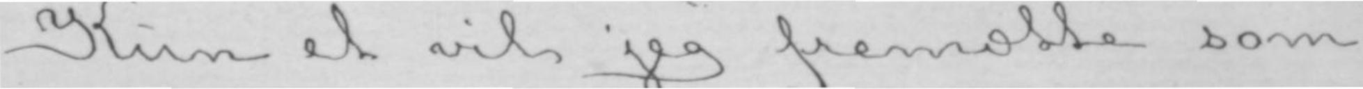Kun et vil jeg fremætte som
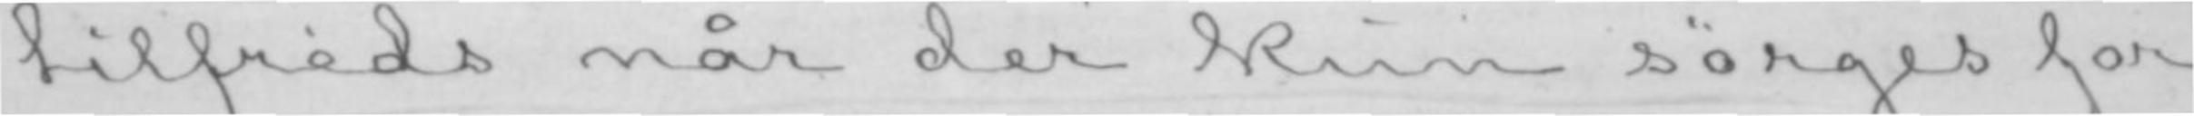tilfreds når der kun sørges for
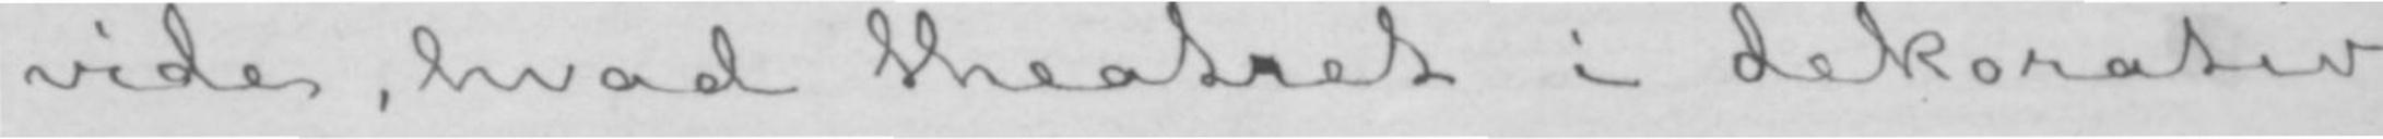vide, hvad theatret i dekorativ
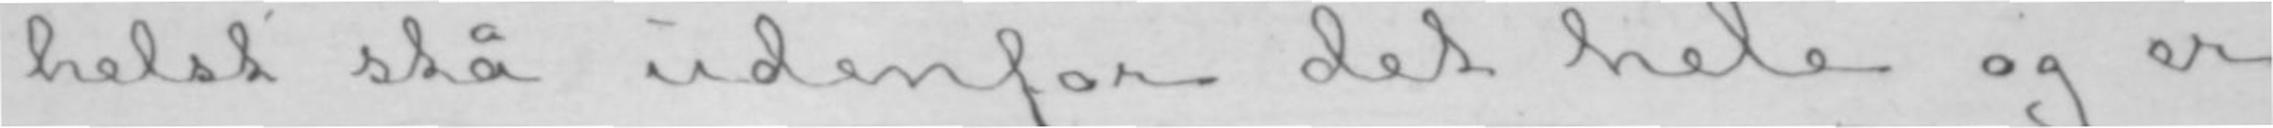helst stå udenfor det hele og er
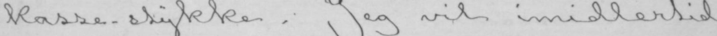kasse-stykke. Jeg vil imidlertid
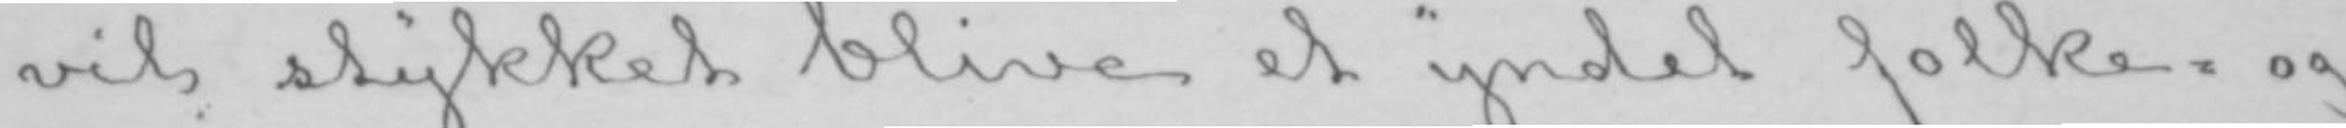vil stykket blive et yndet folke- og
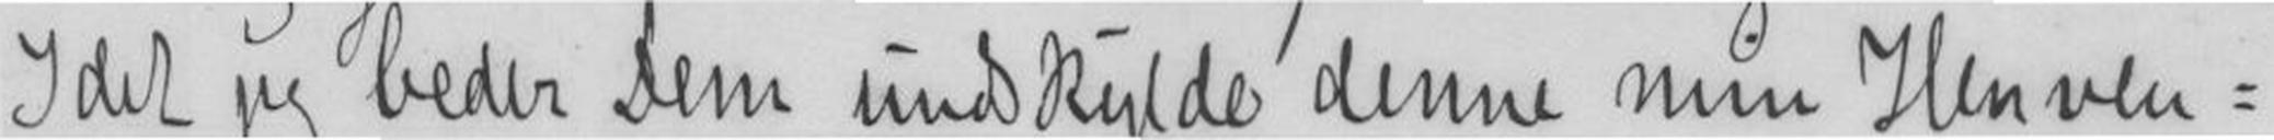Idet jeg beder Dem undskylde denne min Henven-
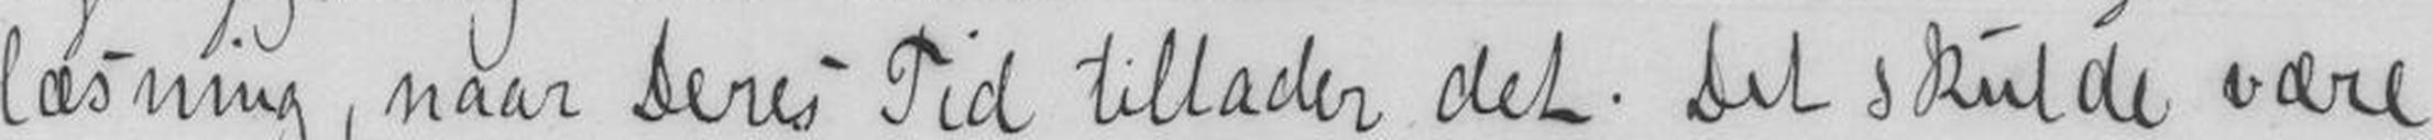læsning, naar Deres Tid tillader det. Det skulde være
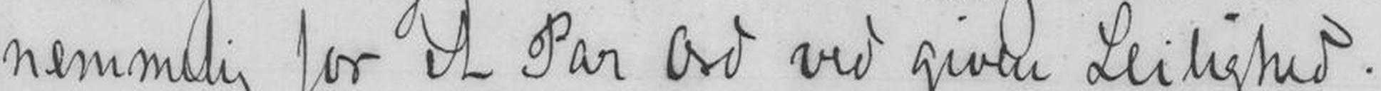nemmelig for et Par Ord ved given Leilighed.
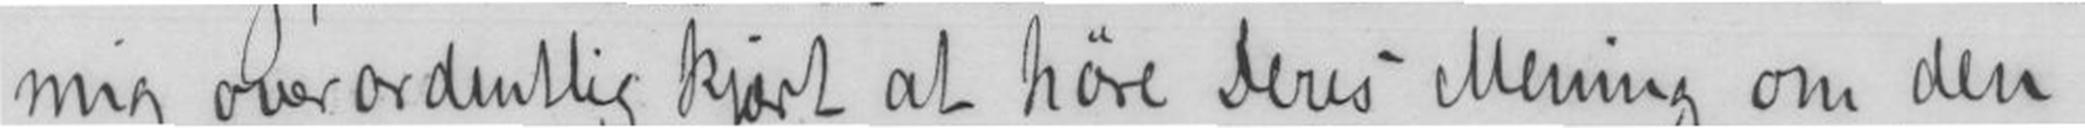mig overordentlig kjært at høre Deres Mening om den,
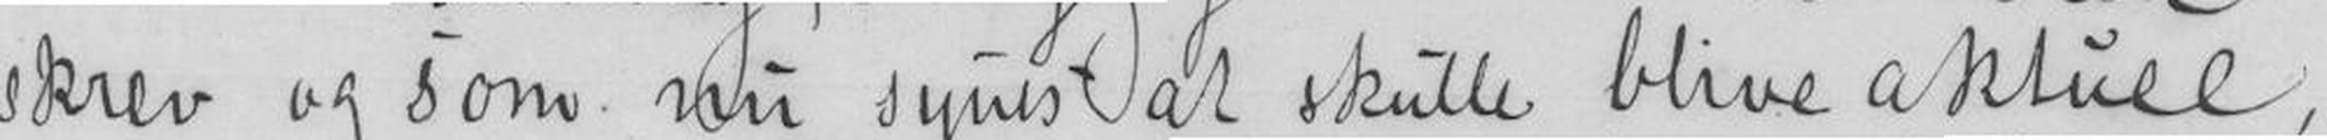skrev og som nu synes at skulle blive aktuel,
